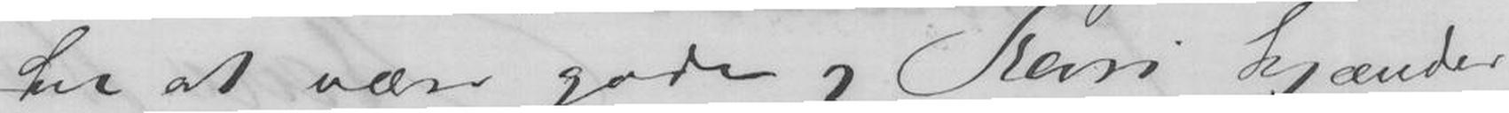til at være gode og Kari kjænder
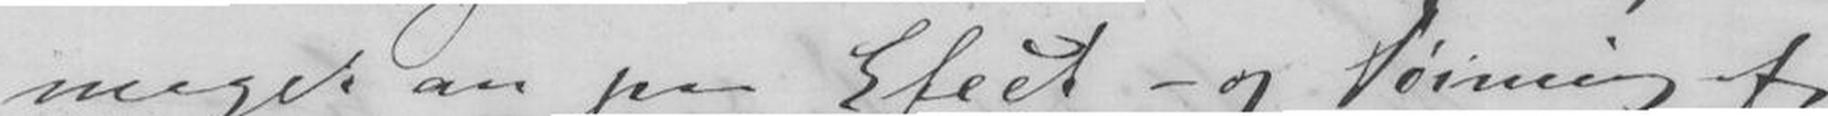meget an paa Efeét - og Tøiming af
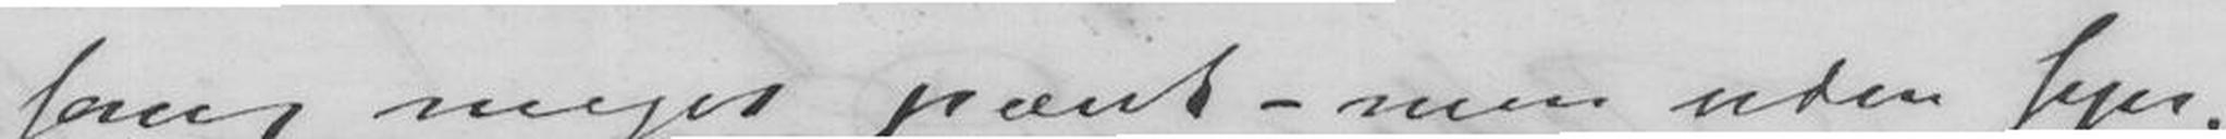sang meget pænt - men uden syn-
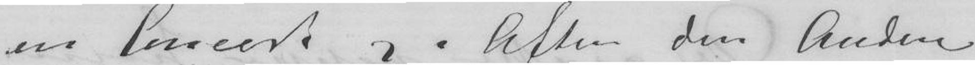en Concert og i Aften den Anden
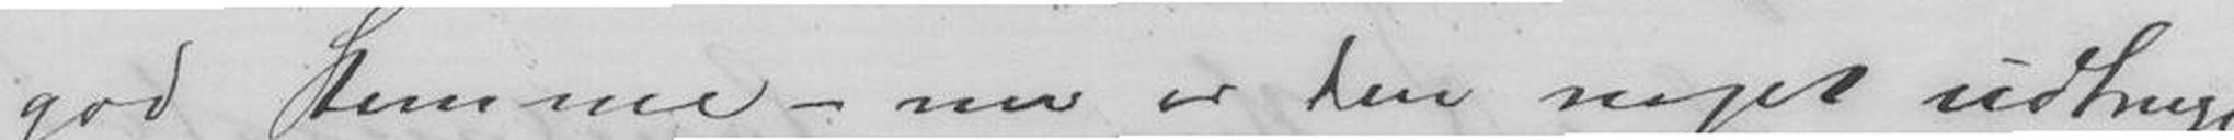god Stemme - nu er den noget udbrugt
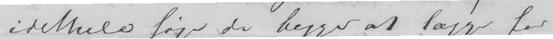idethele søger de begge at lægge for
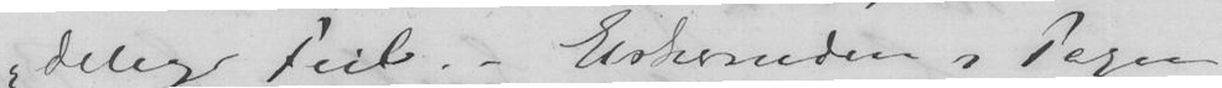delige Feil. - Elskerinden og Tagen
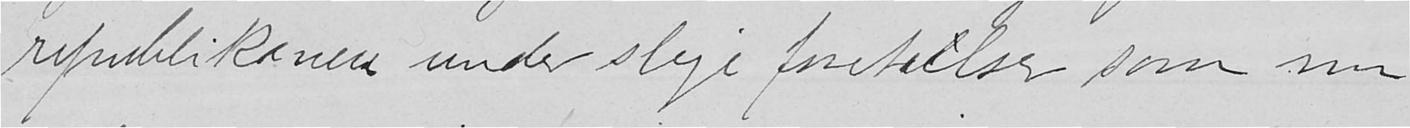republikanere under slige foreteelser som nu
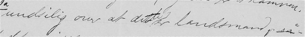undselig over at dette er landsmænd, så-
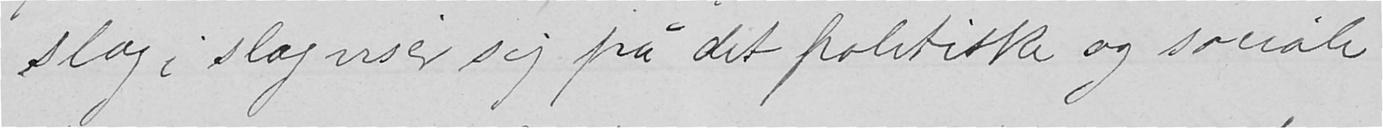slag i slag viser sig på det politiske og sociale
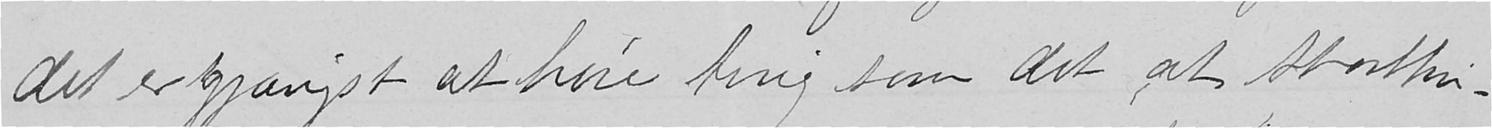det er gjængst at høre ting som det at storthin-
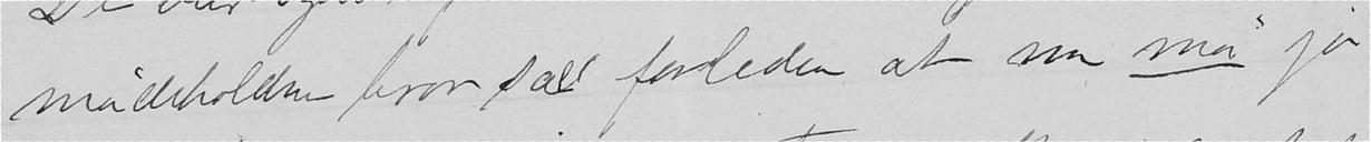mådeholdne bror sae' forleden at nu må jo
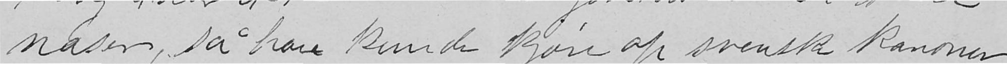næser, så han kunde kjøre op svenske kanoner
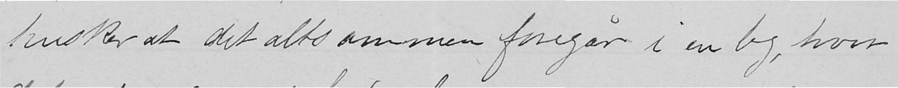husker at det altsammen foregår i en by, hvor
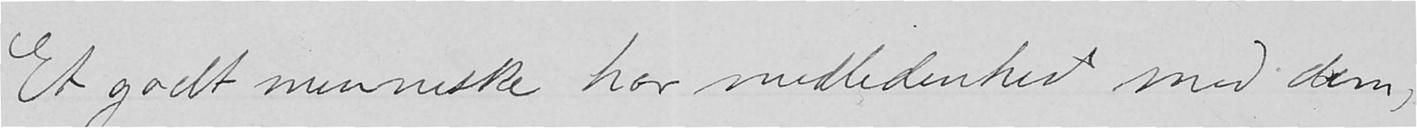Et godt menneske har medlidenhed med dem,
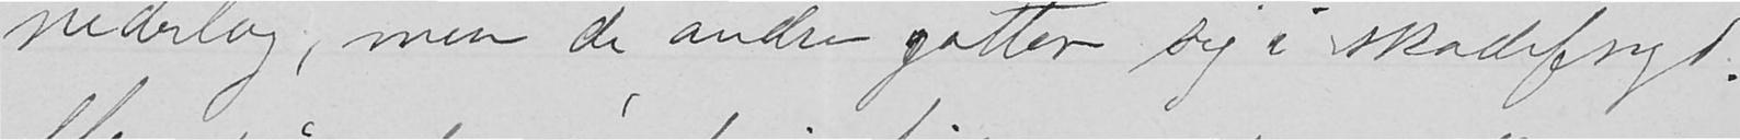nederlag, men de andre gotter sig i skadefryd.
